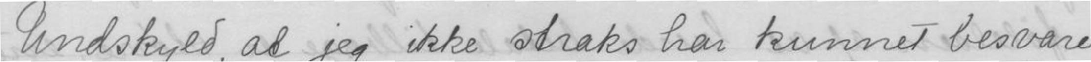Undskyld, at jeg ikke straks har kunnet besvare
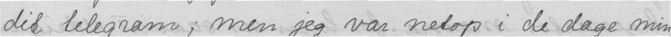det telegram; men jeg var netop i de dage min-
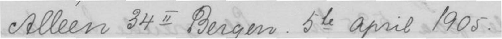Alléen 34II Bergen. 5te april 1905.
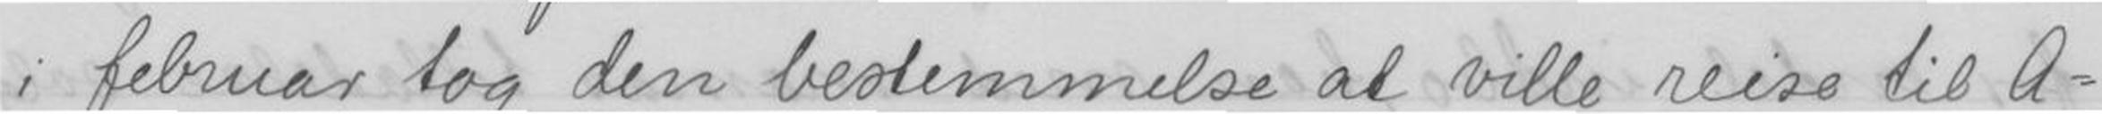i februar tog den bestemmelse at ville reise til A-
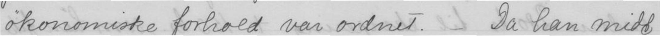økonomiske forhold var ordnet. - Da han midt
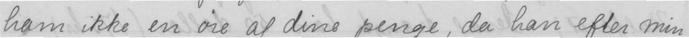ham ikke en øre af dine penge, da han efter min
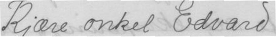Kjære onkel Edvard -
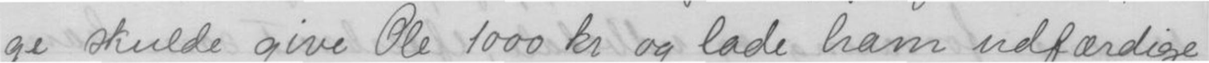ge skulde give Ole 1000 kr og lade ham udfærdige
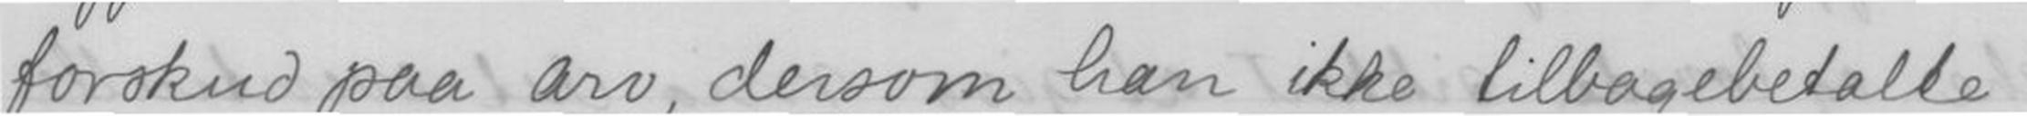forskud paa arv, dersom han ikke tilbagebetalte
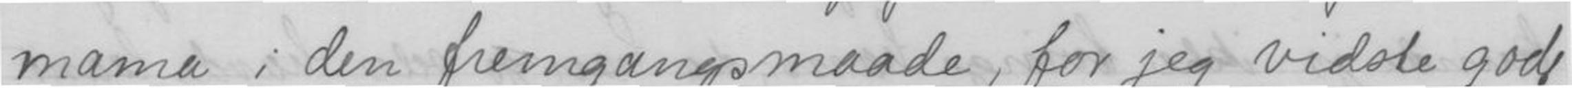mama i den fremgangsmaade, for jeg vidste godt,
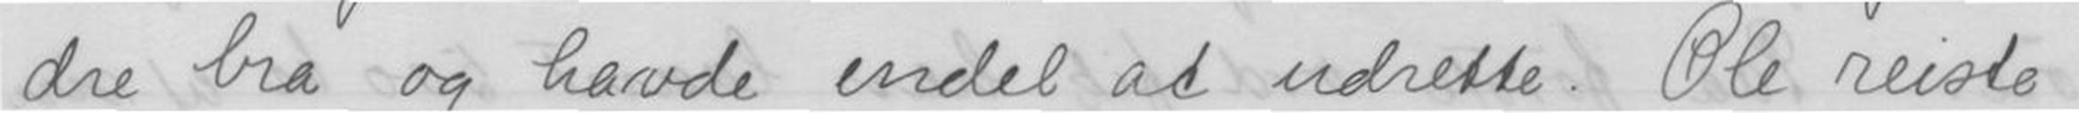dre bra og havde endel at udrette. Ole reiste
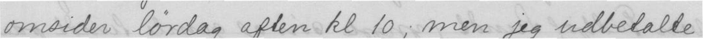omsider lørdag aften kl 10; men jeg udbetalte
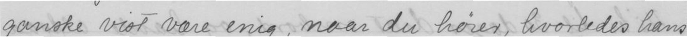ganske vist være enig, naar du hører, hvorledes hans
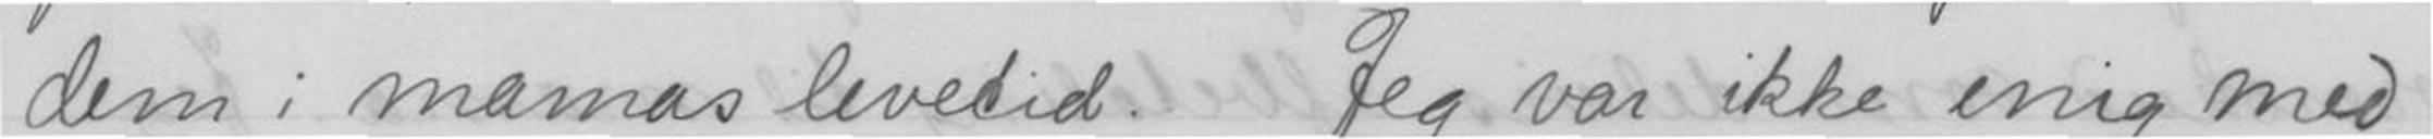dem i mamas levetid. Jeg var ikke enig med
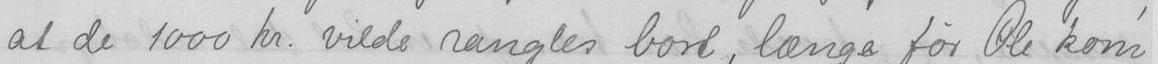at de 1000 kr. vilde rangles bort, længe før Ole kom
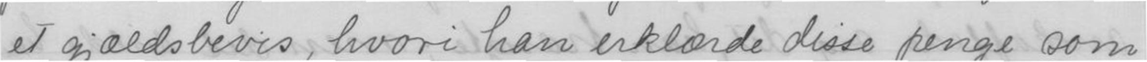et gjældsbevis, hvori han erklærte disse penge som
